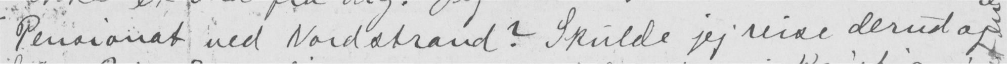Pensionat ved Nordstrand? Skulde jeg reise derud af
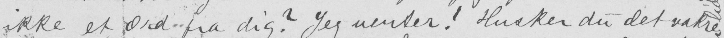ikke et ord fra dig? Jeg venter! Husker du det vakre
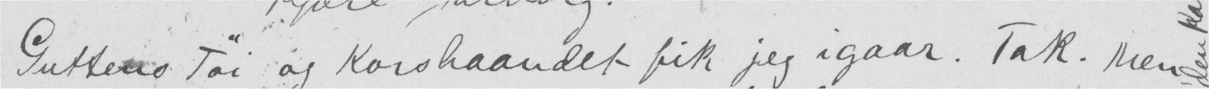Guttens Tøi og Korshaandet fik jeg igaar. Tak. Men
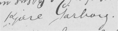Kjære Garborg.
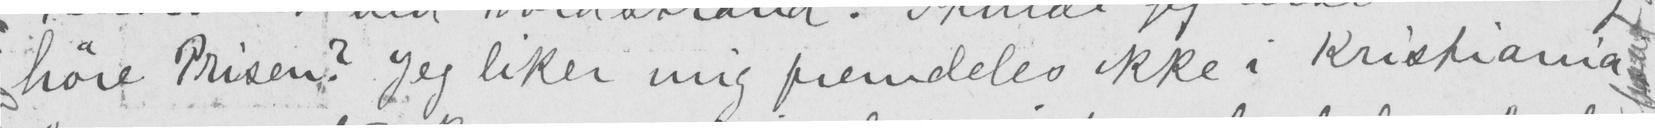høre prisen? Jeg liker mig fremdeles ikke i Kristiana.
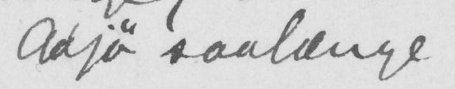Adjø saalænge.
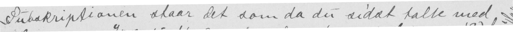Pubskriptionen staar det som da du sidst talte med
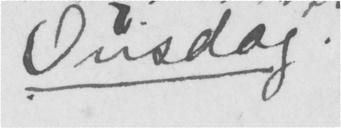Onsdag.
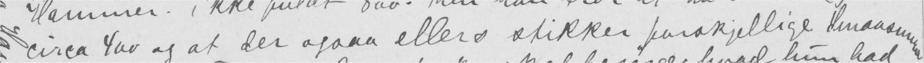circa 400 og at der ogsaa ellers stikker forskjellige smaasummer.
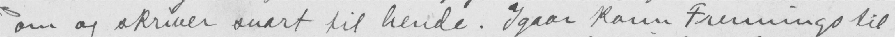om og skriver snart til hende. Igaar kom Frennings til
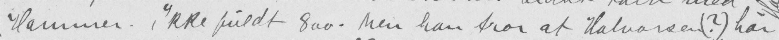Hammer. Ikke fuldt 800. Men han tror at Halvorsen(?) har
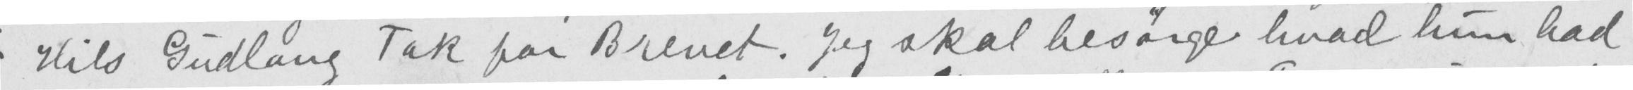Hils Gudlaug tak for brevet. Jeg skal besørge hvad hun bad
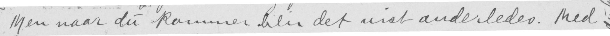Men naar du kommer blir det vist andeledes. Med
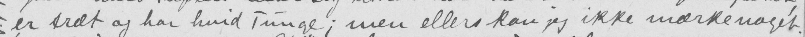er træt og har hvid Tunge; men ellers kan jeg ikke mærke noget.
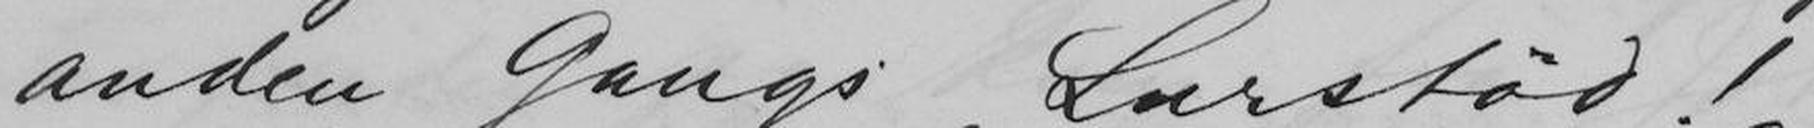anden gangs Lurstød!
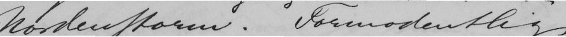Nordenstorm. Formodentlig
